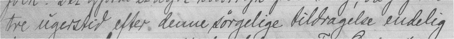tre ugerstid efter denne sørgelige tildragelse endelig
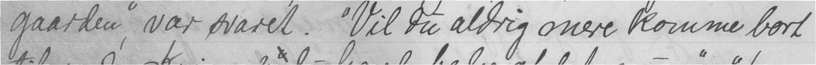gaarden," var svaret. "Vil du aldrig mere komme bort
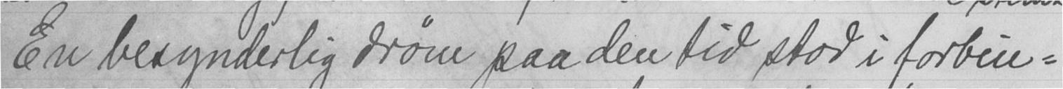En besynderlig drøm paa den tid stod i forbin-
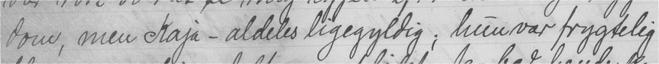dom, men Kaja - aldeles ligegyldig; hun var frygtelig
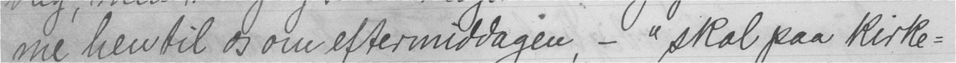me hen til os om eftermiddagen, - "skal paa kirke-
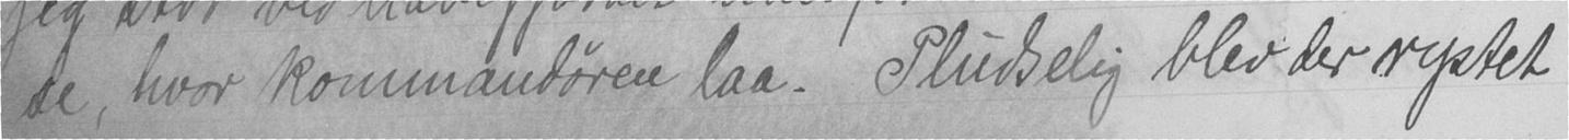se, hvor kommandøren laa. Pludselig blev der rystet
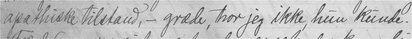apathiske tilstand, - græde, tror jeg ikke, hun kunde.
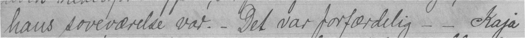hans soveværelse var. - Det var forfærdelig - - Kaja
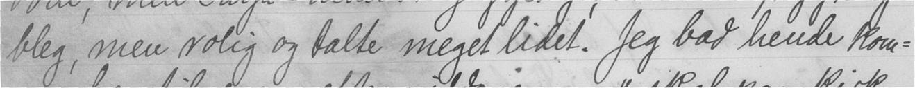bleg, men rolig og talte meget lidet. Jeg bad hende kom-
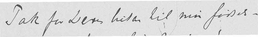Tak for Deres hilsen til min fødsels-
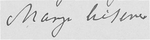Mange hilsener
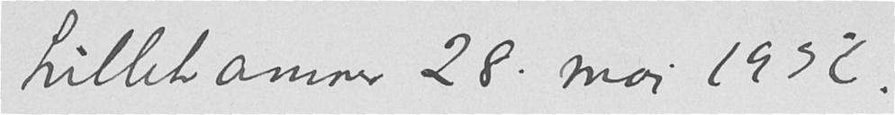Lillehammer 28. mai 1932.
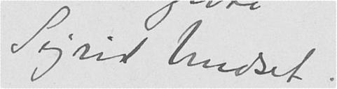Sigrid Undset.
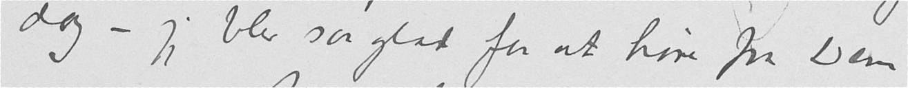dag – jeg blev saa glad for at høre fra Dem!
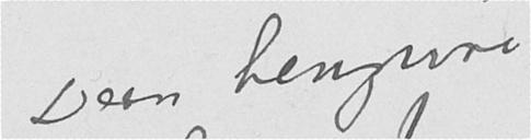Deres hengivne
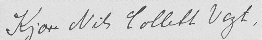Kjære Nils Collett Vogt,
